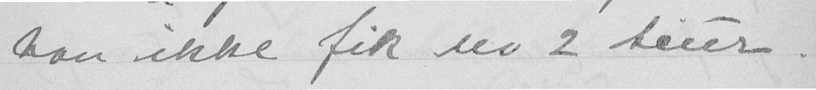han ikke fik no 2 tiur.
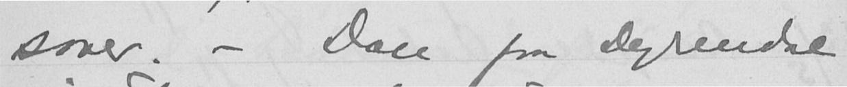sover. - Don paa Dyrendal
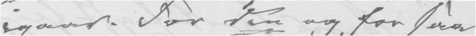igår. For den og for saa
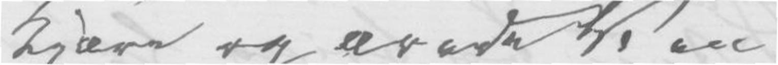Kjære og ærede Ven
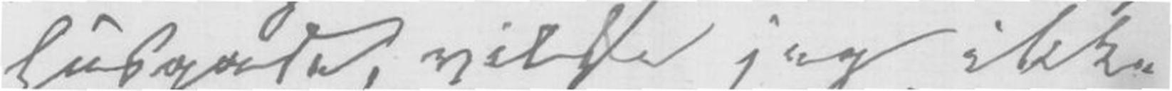husgade, vilde jeg ikke
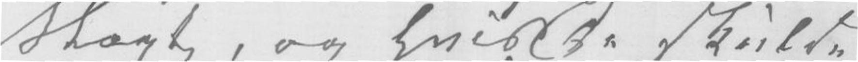Slægt, og hvis De skulde
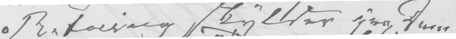Retning skylder jeg Dem
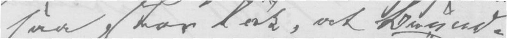saa stor Tak, at Beund-
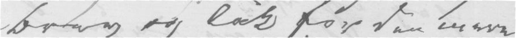Brev og Tak for den mere
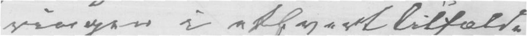ringen i ethvert Tilfælde
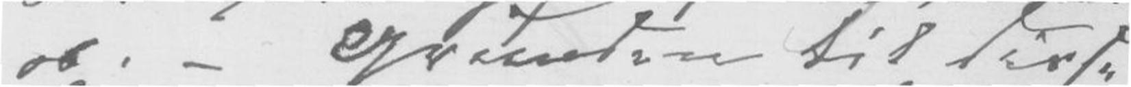os.- Grunden til disse
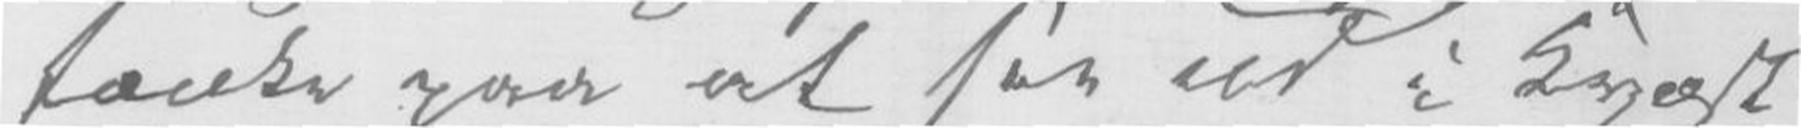tænke paa at see ud i Kvæst-
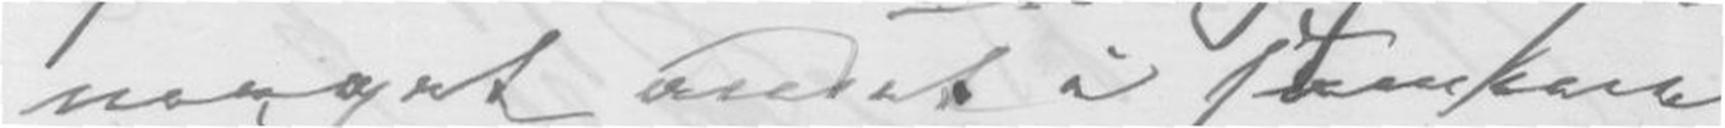meget andet i samme
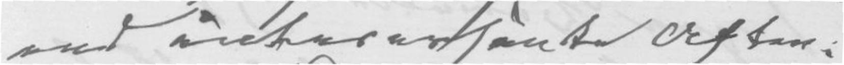end interessantere Aften.
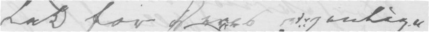Tak for Deres venlige
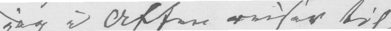jeg i Aften reiser til
